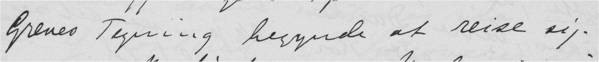Greves Tegning begynde at reise sig.
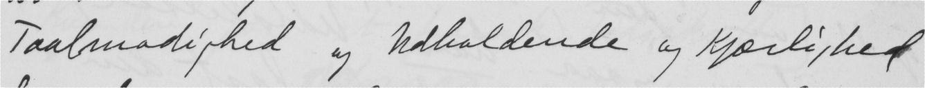Taalmodighed og Udholdende og Kjærlighed
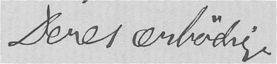Deres ærbødige
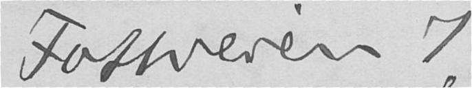Fossveien 7,
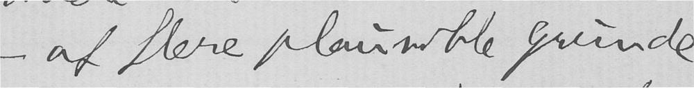- af flere plausible Grunde.
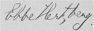Ebbe Hertzberg.
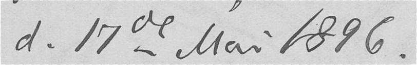d. 17de Mai 1896.
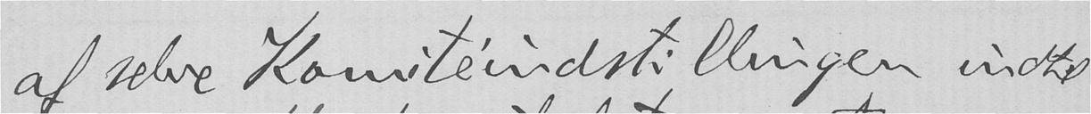af selve Komitéindstillingen indtil
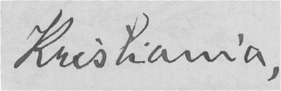Kristiania,
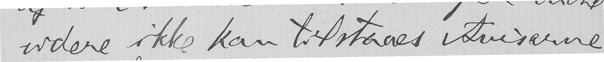videre ikke kan tilstaaes Aviserne,
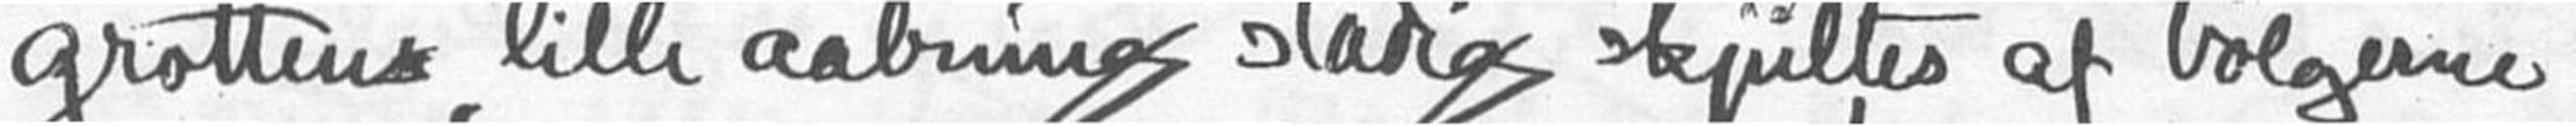grottens lille aabning stadig skjultes af bølgerne
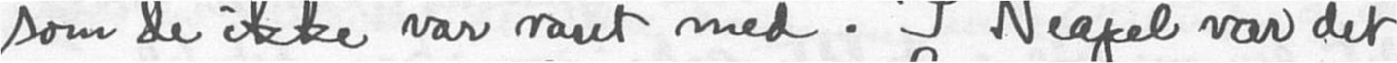som de ikke var vant med. I Neapel var det
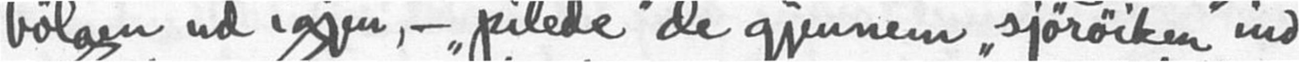bølgen ud igjen,- "pilede" de gjennem "sjorøiken" ind
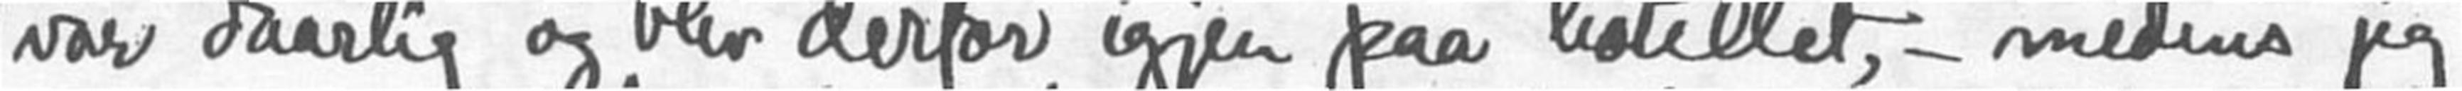var daarlig og blev derfor igjen paa hotellet, - medens jeg
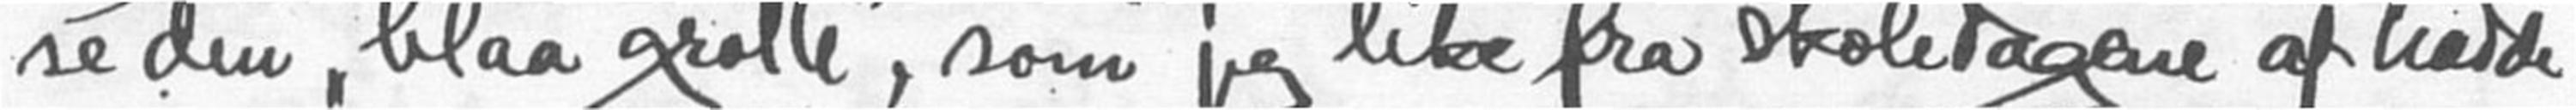se den "blaa grotte", som jeg like fra skoledagene af hadde
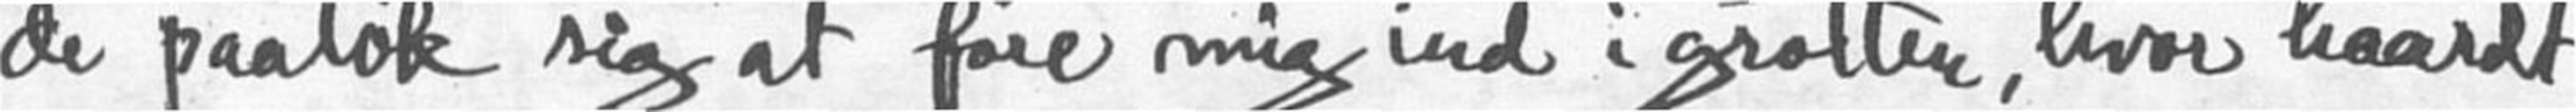de paatok sig at føre mig ind i grotten, hvor haardt
